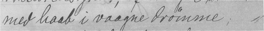med haab i vaagne drømme;
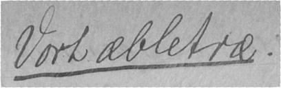Vort æbletræ.
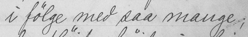i følge med saa mange;
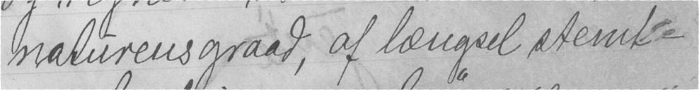naturens graad, af længsel stemt -
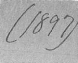(1897)
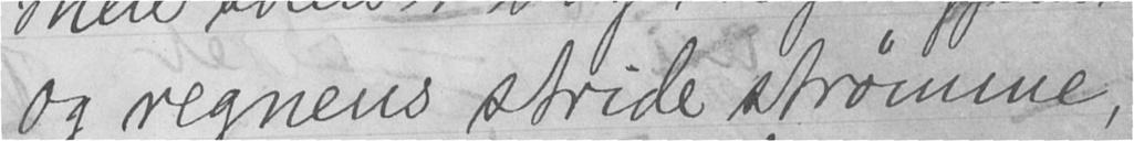og regnens stride strømme,
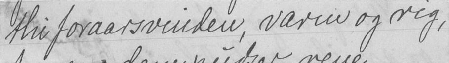thi foraarsvinden, varm og rig,
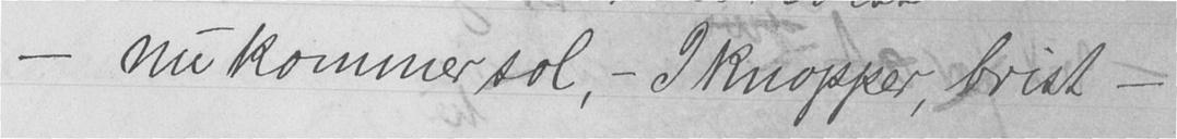- nu kommer sol, - I knopper, brist -
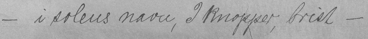- i solens navn, I knopper, brist -
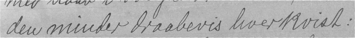den minder draabevis hver kvist:
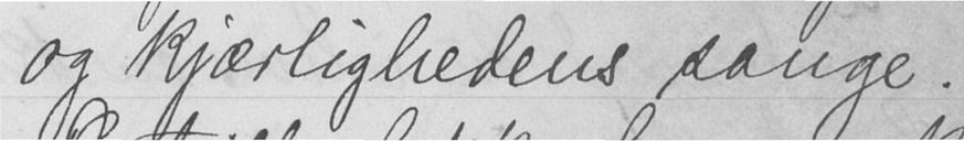og kjærlighedens sange.
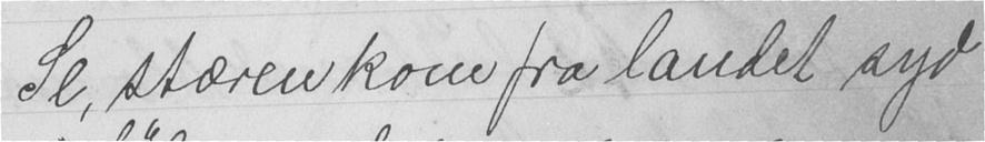Se, stæren kom fra landet syd
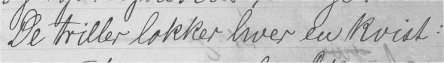De triller lokker hver en kvist:
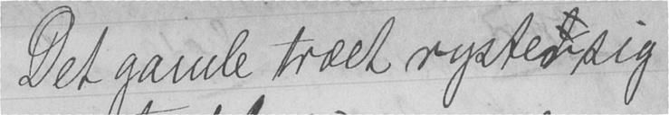Det gamle træet rysted sig
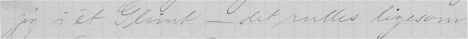jeg i ét GIimt - det rulles ligesom
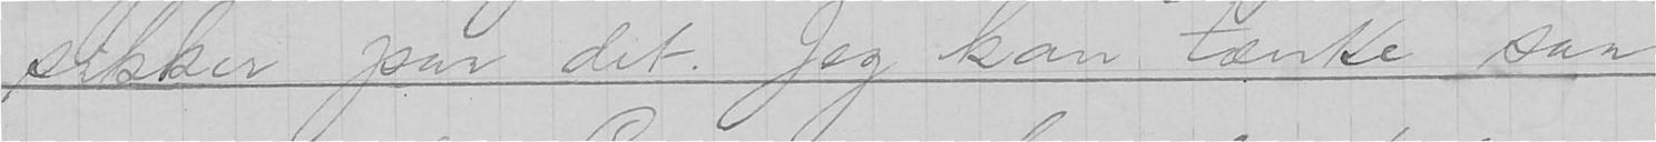sikker paa det. Jeg kan tænke saa
